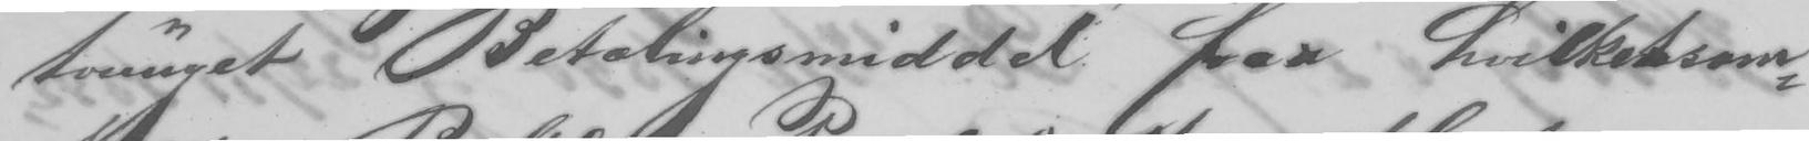tvunget Betalingsmiddel for hvilketsom-
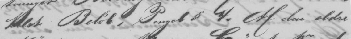helst Beløb, Pengel §4. Af den ældre
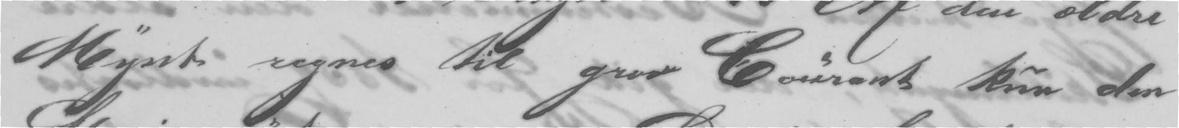Mynt regnes til grov Courant kun den
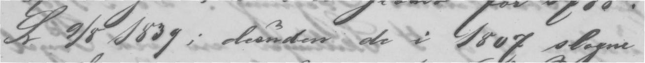Fr 9/8 1839; desuden de i 1807 slogne
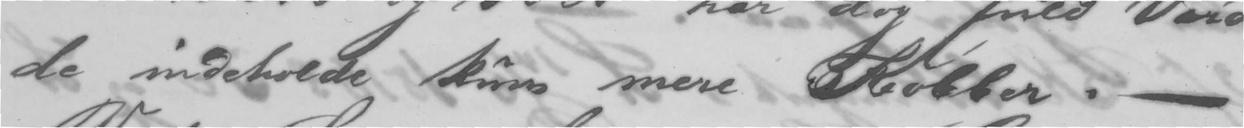de indeholde kun mere Kobber. -
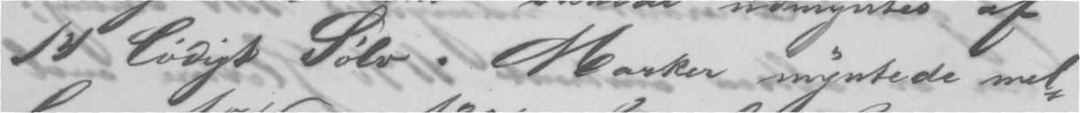14 løligt Sølv. Marken myntede mel-
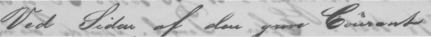Ved Siden af den grove Cournant
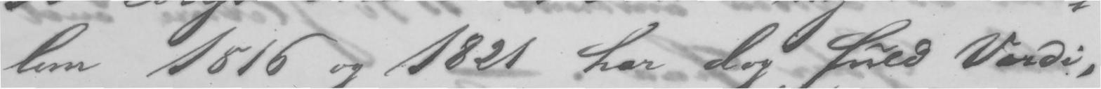lem 1816 og 1821 har dog fuld Værdi,
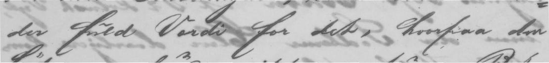der fuld Værdi for det, hvorpaa den
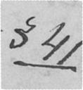§ 41
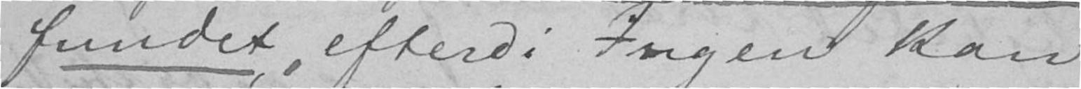fundet, efterdi Ingen kan
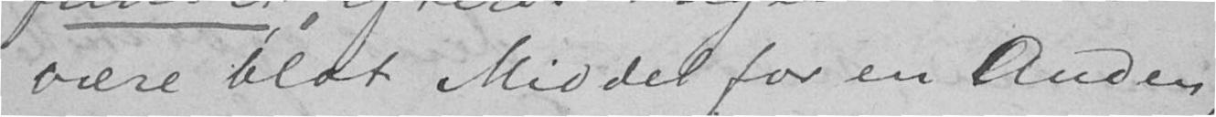være blot Middel for en Anden;
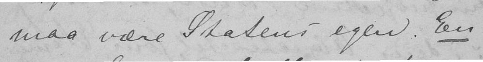maa være Statens egen. En-
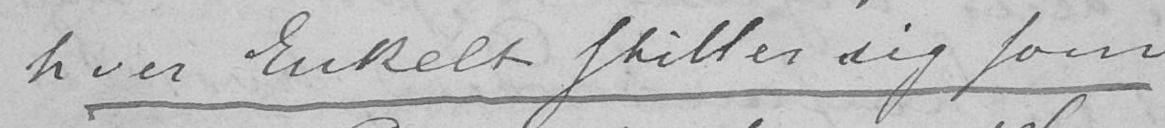hver Enkelt stiller sig som
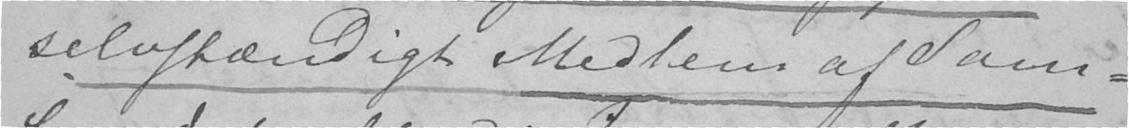selvstændigt Medlem af Sam-
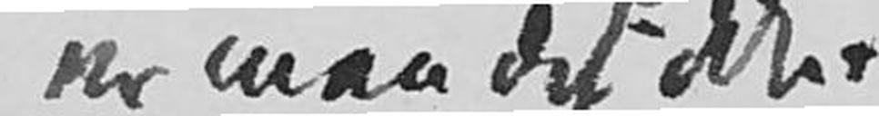er man det ikke.
